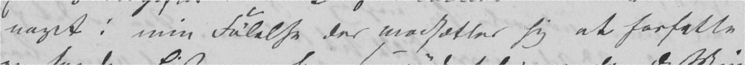noget i min Følelse der modsætter sig at forfatte
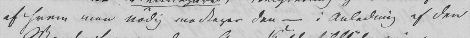af hvem man nødig modtager den – i Anledning af den
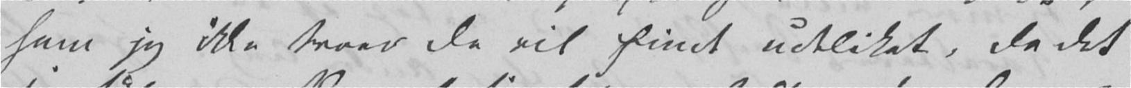som jeg ikke troer De vil finde udelikat, da det
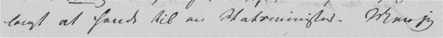langt at sende til en Statsminister. Men jeg
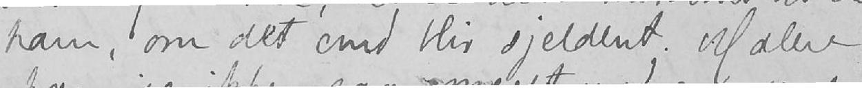ham, om det emd blir sjeldent. Malere
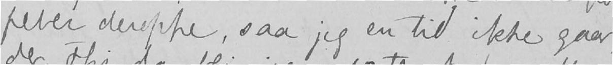feber deroppe, saa jeg en tid ikke gaar,
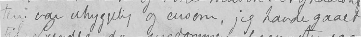ten var uhyggelig og ensom, jeg havde gaaet
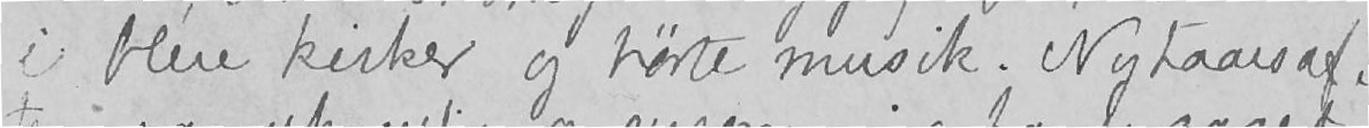i flere kirker og hørte musik. Nytaarsaf-
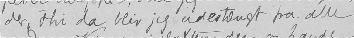der, thi da blir jeg udestængt fra alle
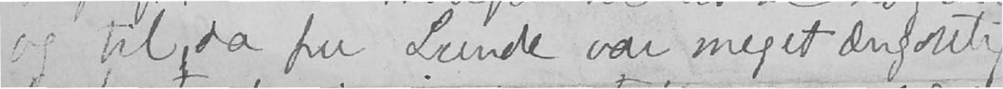og til, da fru Lunde var meget ængstelig,
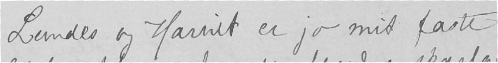Lundes og Harriet er jo mit faste
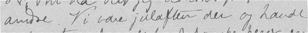andre. Vi vare julaften der og havde
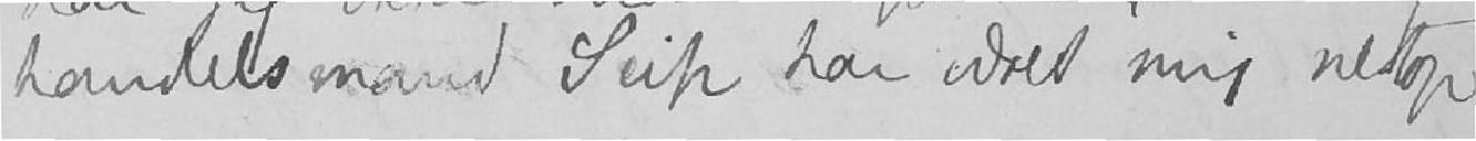handelsmand Seip har være mig netop
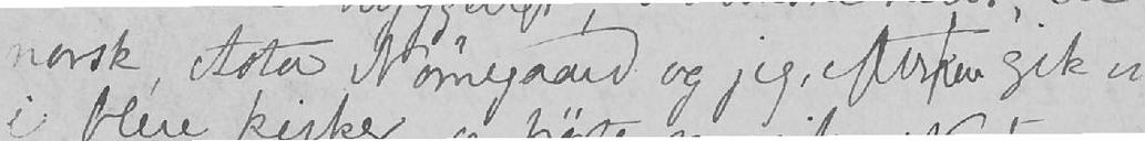norsk, Asta Nørregaard og jeg, efterpaa gik vi
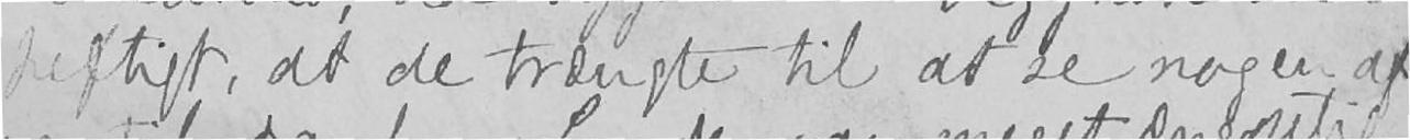heftigt, at det trængte til at se nogen af
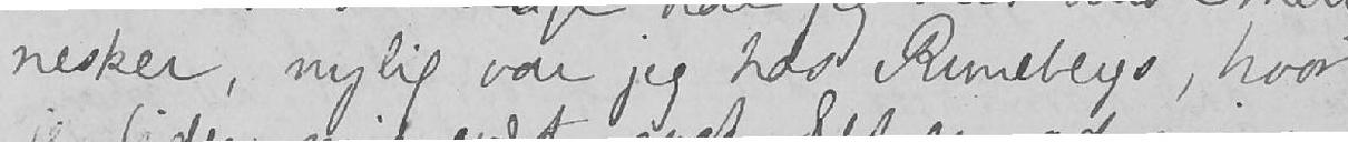nesker, nylig var jeg hos Runebergs, hvor
